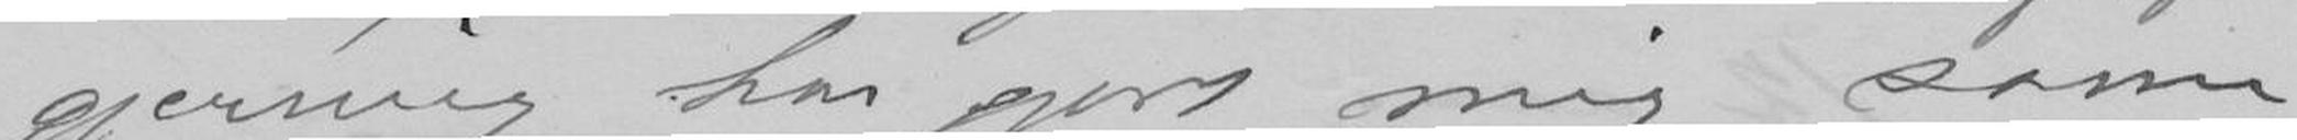gjerning har gjort mig som
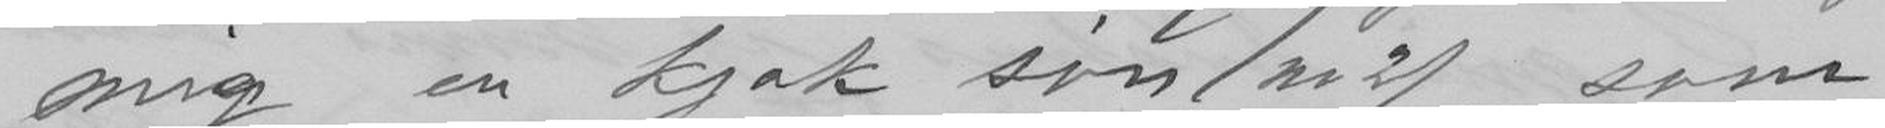mig en kjek søn/nr 2/, som
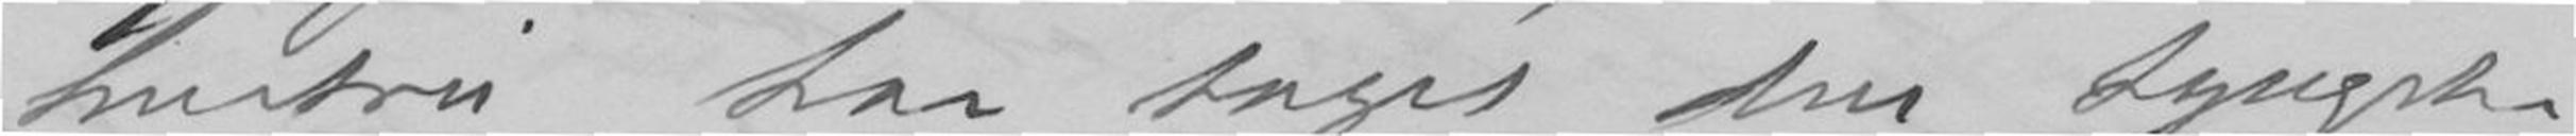hustru har taget den tyngste
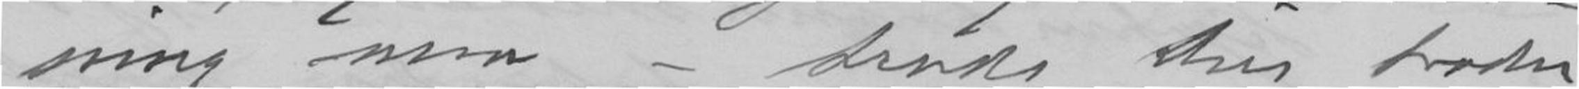ning maa - trods Din broder
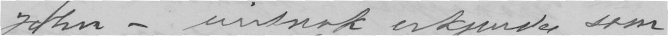John - vistnok erkjenelse som
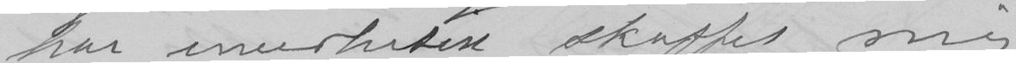har imidlertid skaffet mig
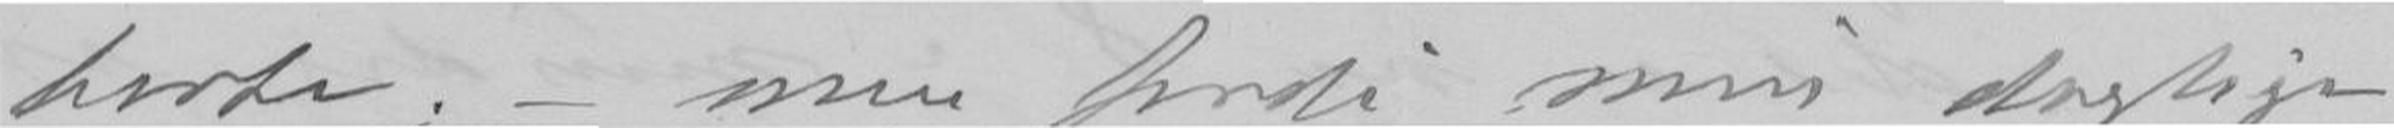borte; men fordi min dygtige
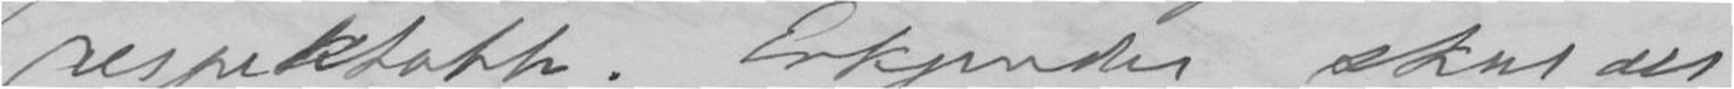respektatte. Erkjender skal det
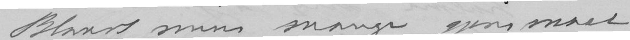Blandt mine mange gjøremaal
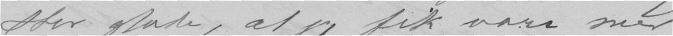stor glæde, at jeg fik være med,
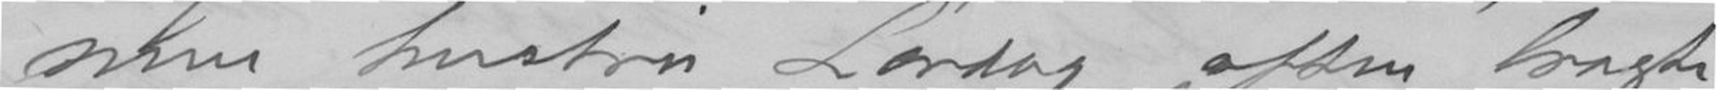min hustru Lørdag aften bragte
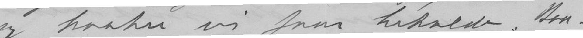jeg haaber vi faar beholde. Baa-
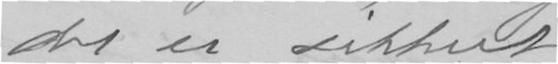det er sikkert.
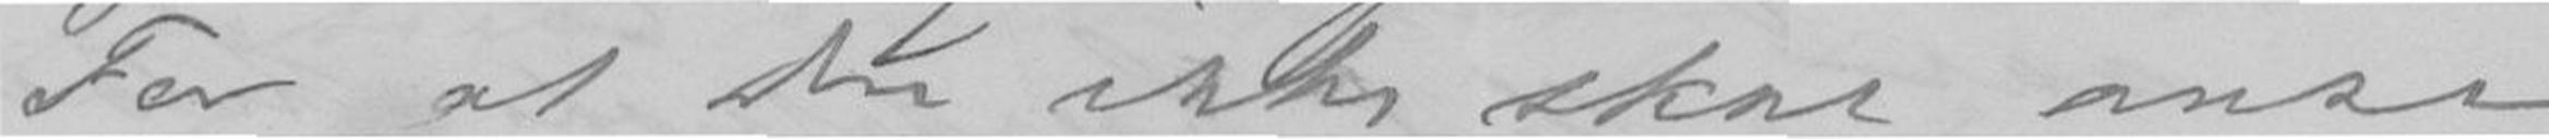For at du ikke skal anse
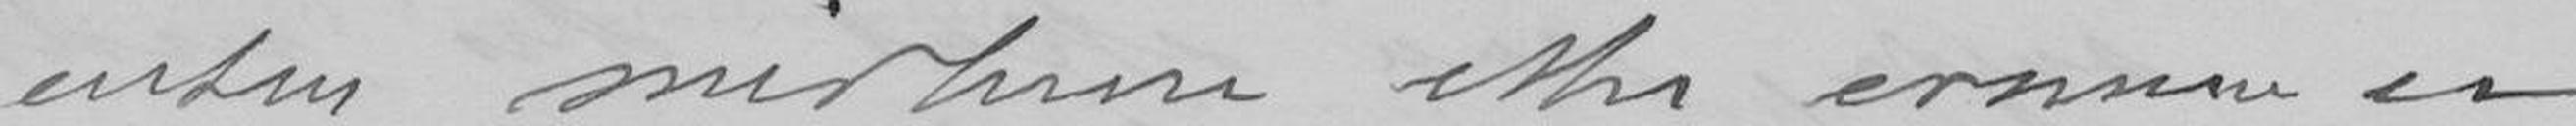enten midlerne eller evnerne er
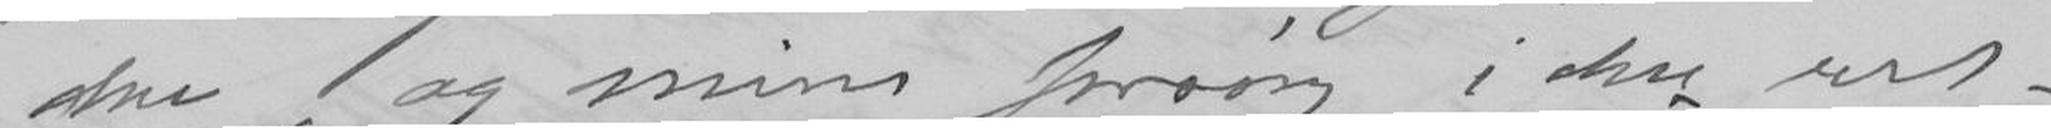den, og mine forsøg i den ret-
